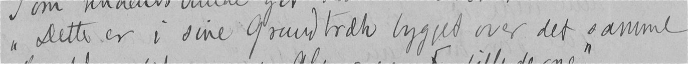"Dette er i sine Grundtræk bygget over det samme
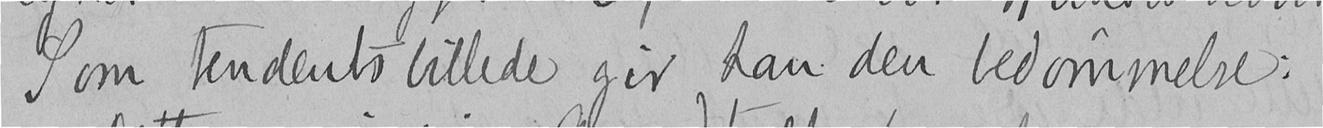Som tendentsbillede gir han den bedømmelse:
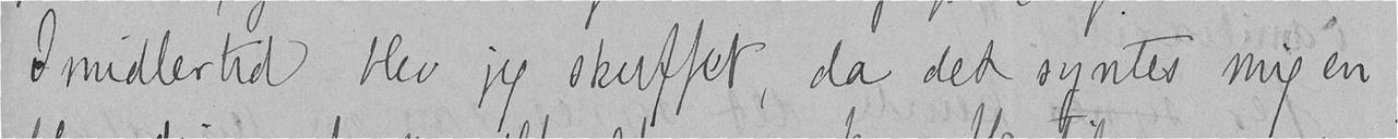Imidlertid blev jeg skuffet, da det syntes mig en
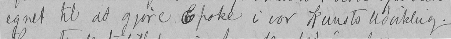egnet til at gjøre Epoke i vor Kunsts Udvikling.
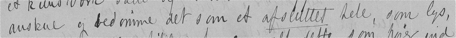anskue og bedømme det som et afsluttet hele, som lys,
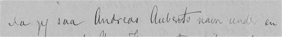Da jeg saa Andreas Auberts navn under en
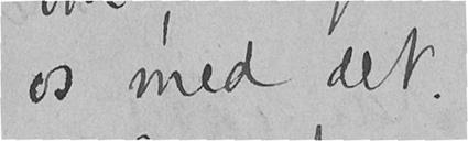os med det.
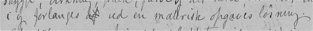i og forlanges af ved en malerisk opgaves løsning.
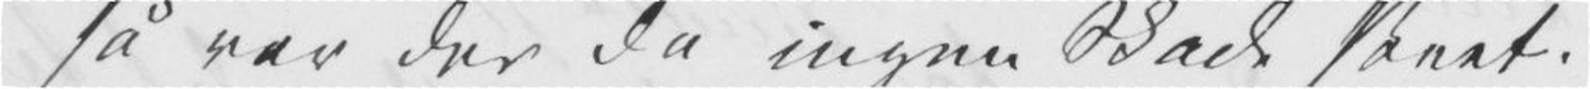så var der da ingen Skade skeet.
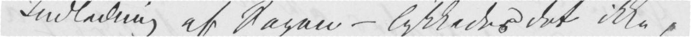Indledning af Sagen – lykkedes det ikke,
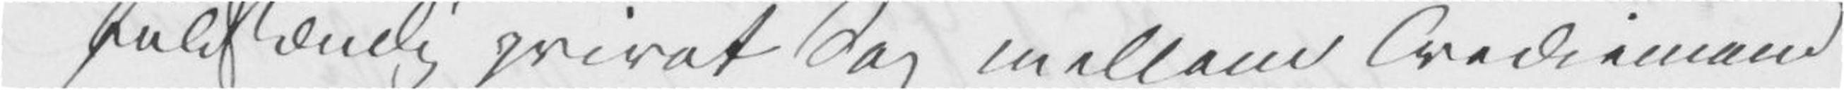fuldstændig privat Sag mellem Trediemand
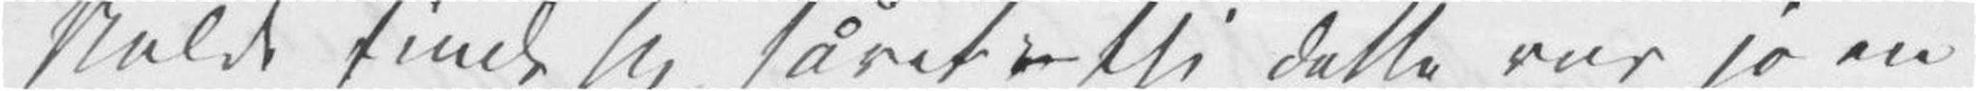skulde finde sig såret – thi dette var jo en
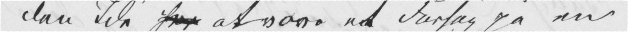den Idé <gap /></del> at vove et Forsøg på en
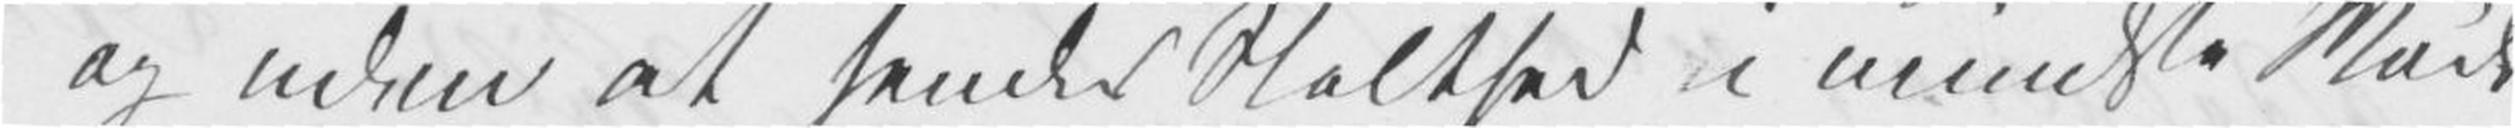og uden at hendes Stolthed i mindste Måde
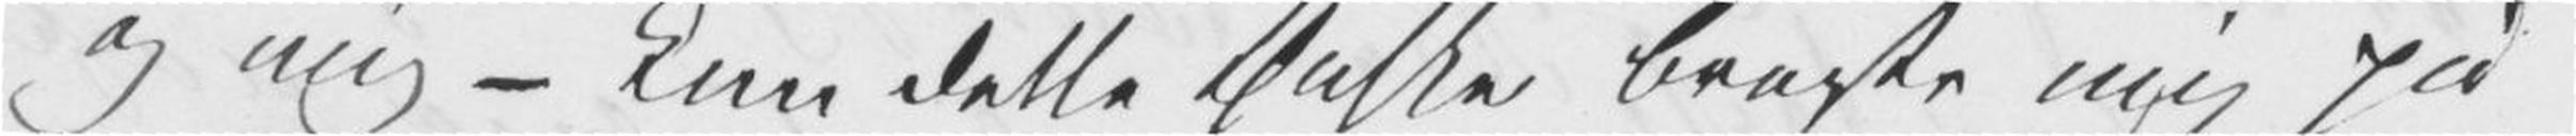og mig – kun dette Ønske bragte mig på
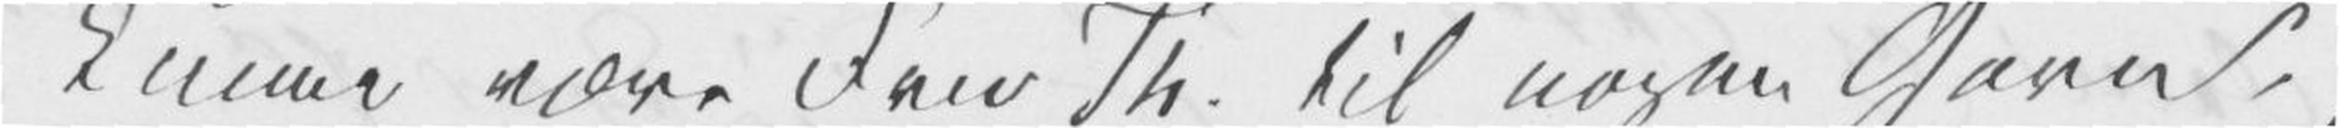kunne være Fru Th. til nogen Gavn,
